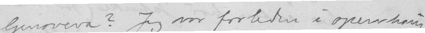Genoveva? Jeg var forleden i operahaus
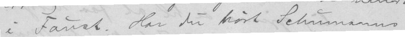i Faust. Har du hørt Schumanns
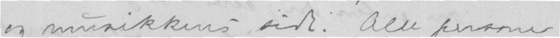og musikkens side. Alle personer
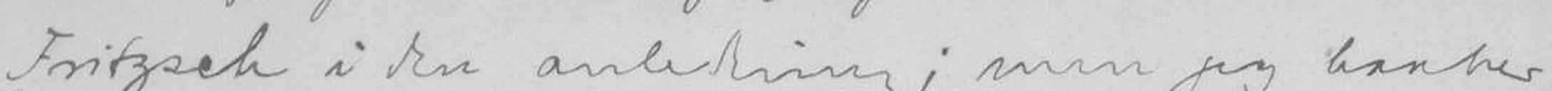Fritzsch i den anledning; men jeg haaber
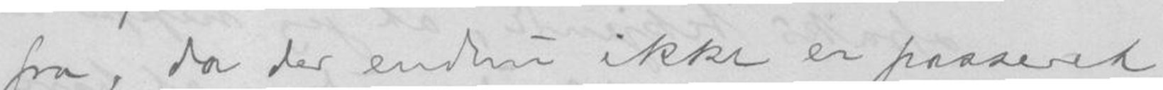fra, da der endnu ikke er passeret
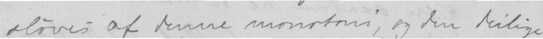sløves af denne monotoni, og den deilige
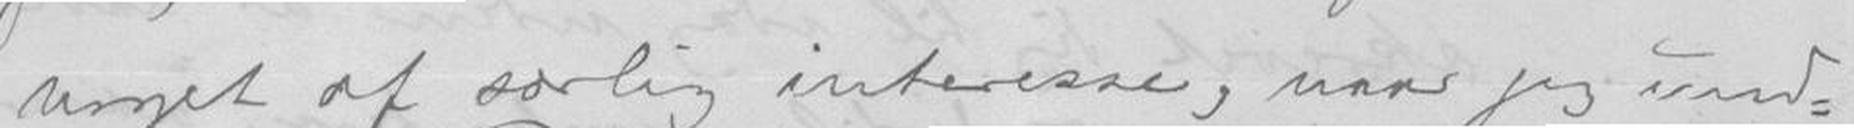noget af særlig interesse, naar jeg und-
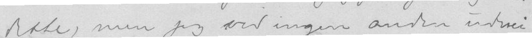dette, men jeg ved ingen anden udvei
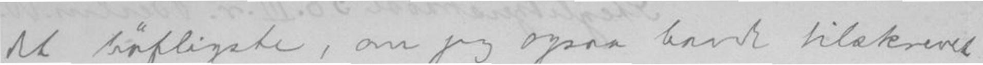det høfligste, om jeg ogsaa havde tilskevet
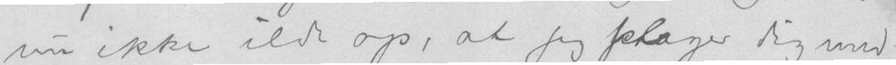nu ikke ilde op, at jeg pager dig med
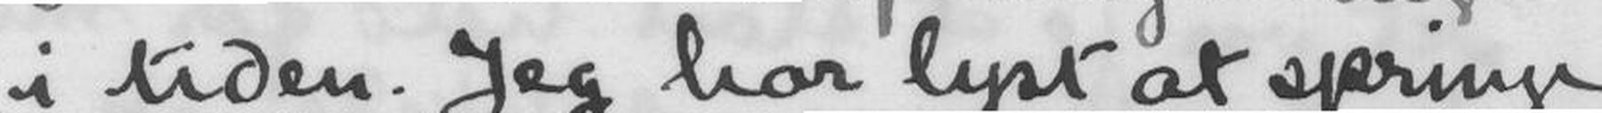i tiden. Jeg har lyst at springe
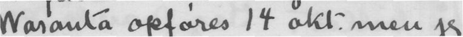Waranta opføres 14 okt. men jeg
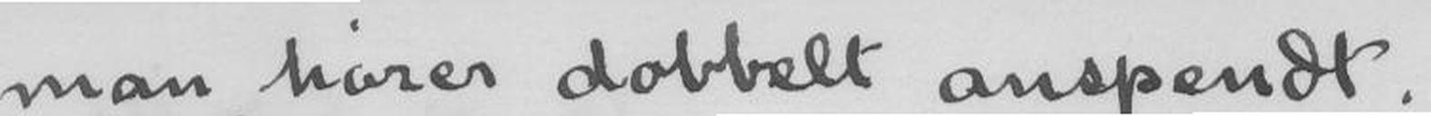man hører dobbelt anspendt.
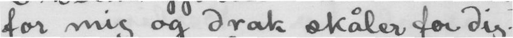for mig og drak skåler for Dig.
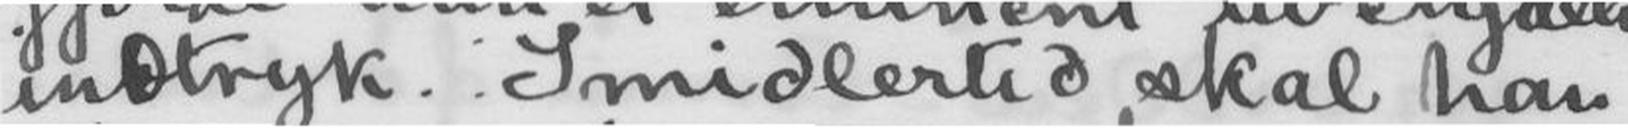indtryk.: Imidlertid skal han
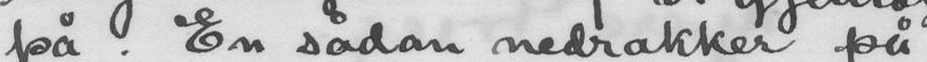på. En sådan nedrakker på
